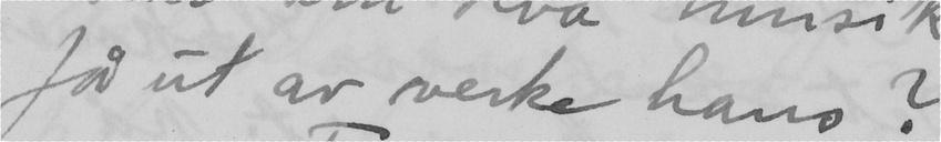få ut av verke hans?
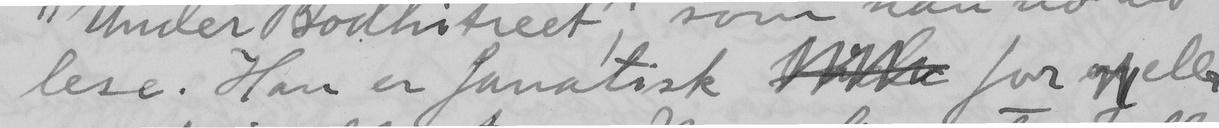lese. Han er fanatisk  for ella
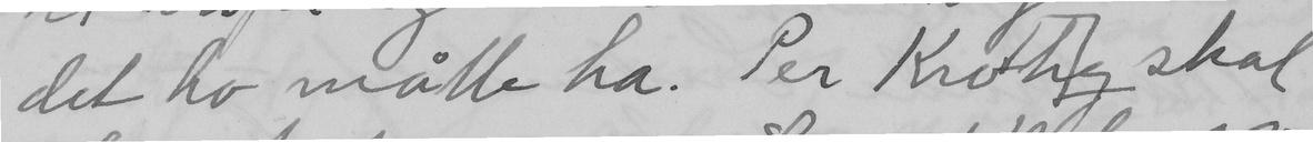det ho måtte ha. Per Krohg skal
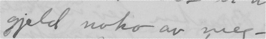gjeld noko av meg -
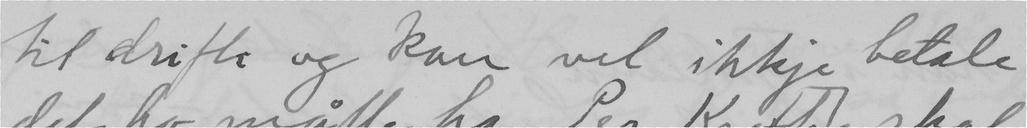til drifta og kan vel ikkje betale
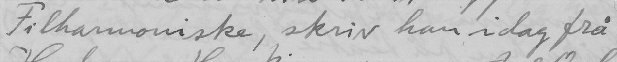Filharmoniske, skriv han idag frå
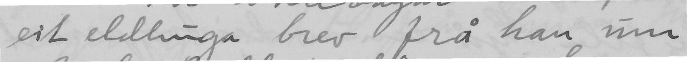eit eldhuga brev frå han um
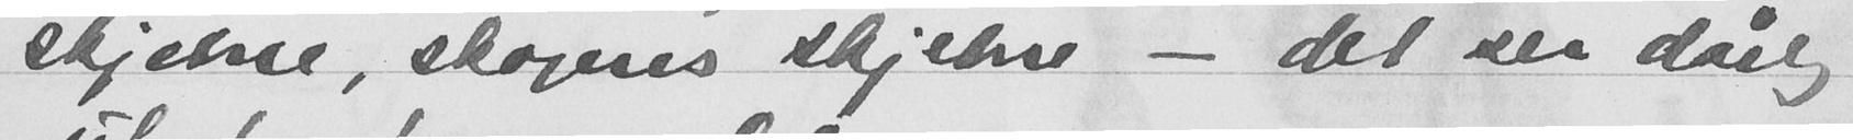skjebne, skogens skjebne - det ser dårlig
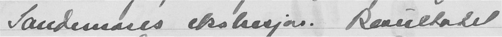Sandemoses eksklusjon. Resultatet
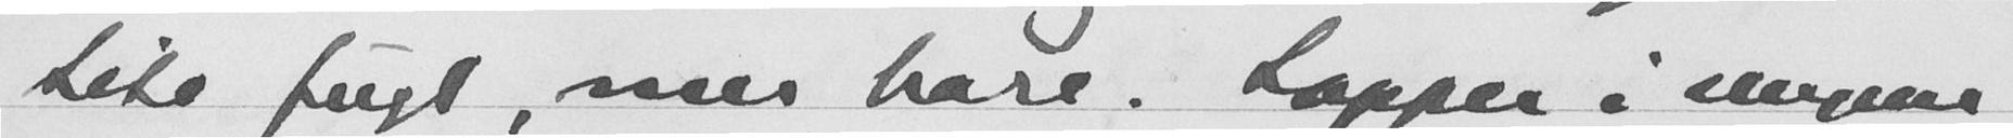Lite frugt, men hare. Lopper i sengene
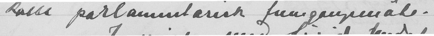kalle parlamentarisk fremgangsmåte.
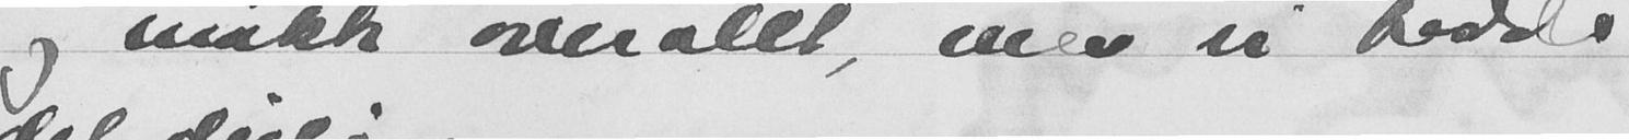og møkk overalt, men vi hadde
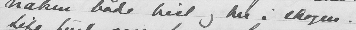naken både hist og her i skogen.
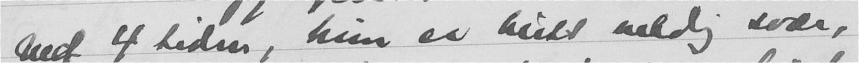ved 4 tiden, hun er blitt veldig svær,
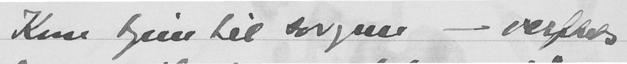Kom hjem til sorgen - verftes
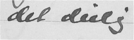det deilig.
